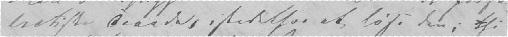lectiske Traade, istedetfor at løse den; thi
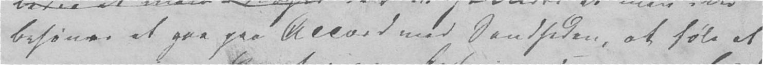behøver at gaa paa Accord med Sandheden, at føle at
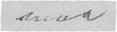maa
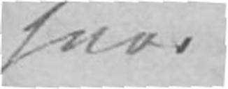hvor
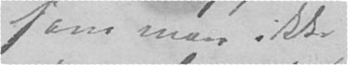som man ikke
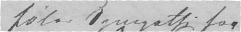føler Sympathi for
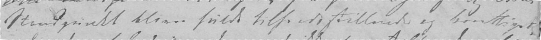Standpunkt bliver fuldt tilfredsstillende og berettiget,
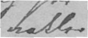heller
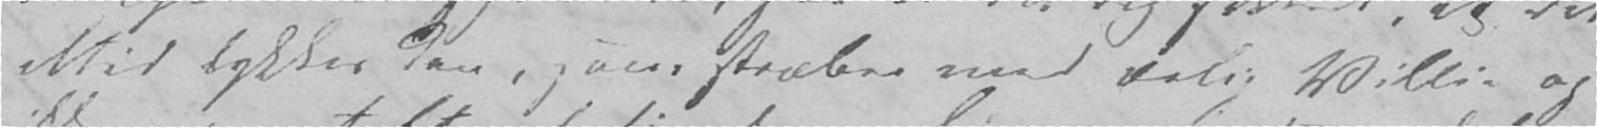altid lykkes den, som stræber med ærlig Villie og
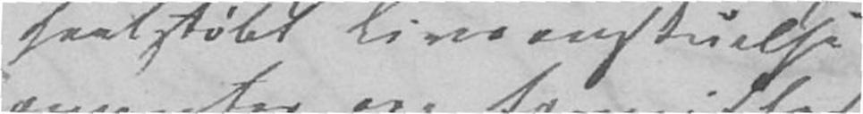faststøbt Livsanskuelse
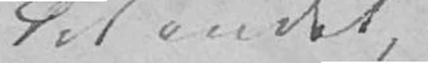det andet,
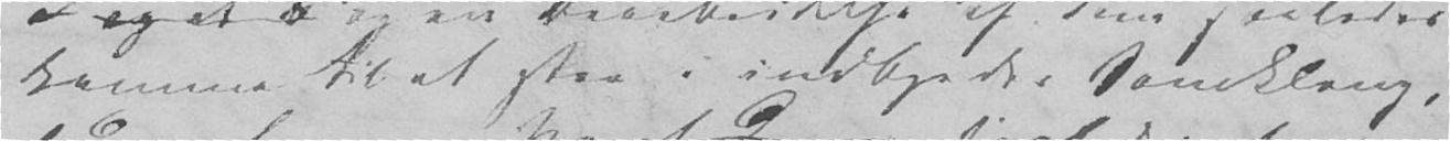komme til at staa i indbyrdes Samklang,
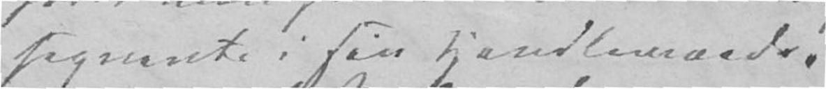seqvents i sin Handlemaade.
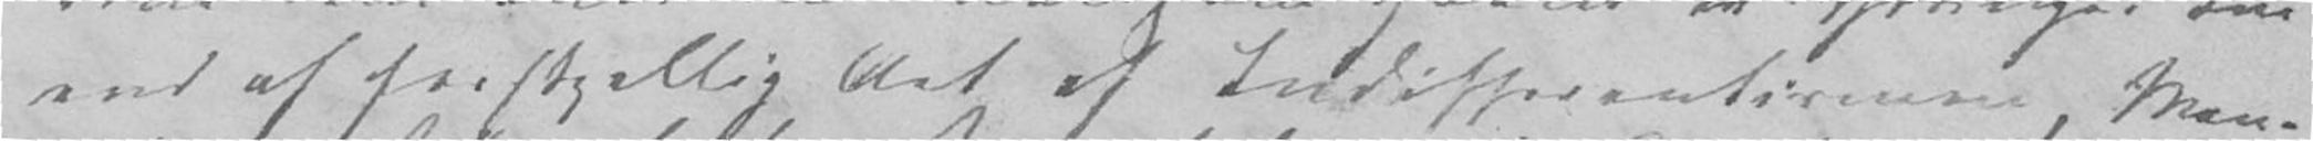end af forskjellig Art af Indifferentieren, Man-
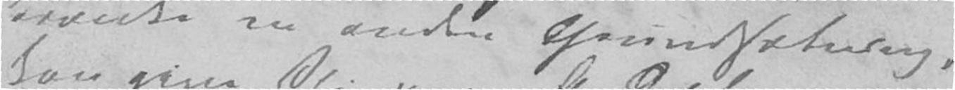krænke en anden Grundsætning,
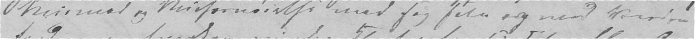Mismod og Misfornøielse med sig selv og med Verden
